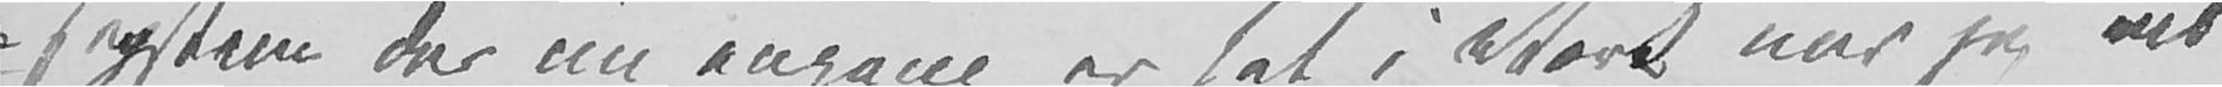system der nu engang er sat i Værk når jeg vil
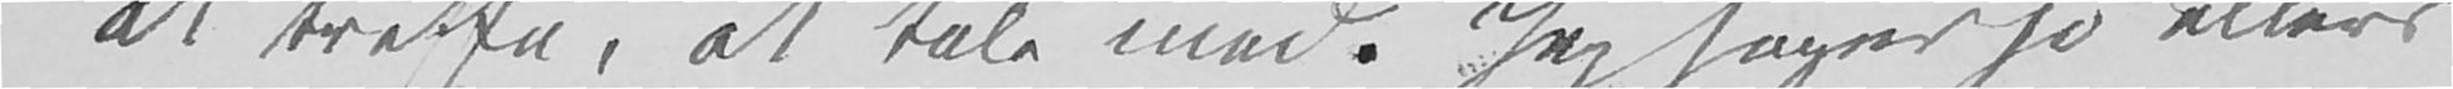at treffe, at tale med. Jeg søger jo ellers
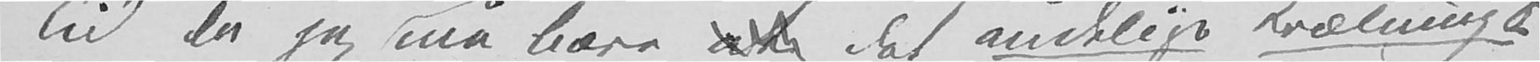Tid da jeg må bære at det åndelige Kvælnings¬
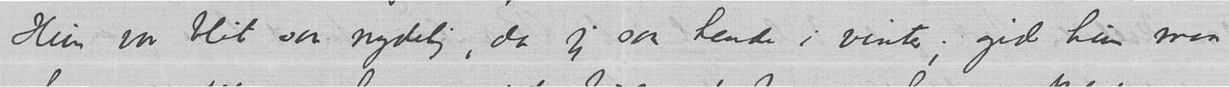Hun var blit saa nydelig, da jeg saa hende i vinter; gid hun maa
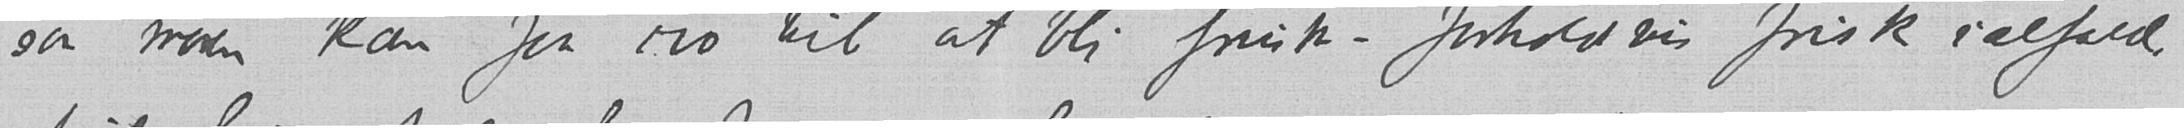saa moren kan faa ro til at bli frisk – forholdvis frisk ialfald,
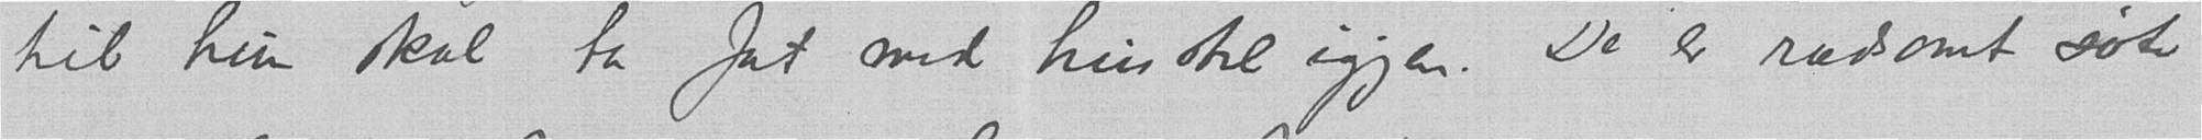til hun skal ta fat med husstel igjen. De er rædsomt søte
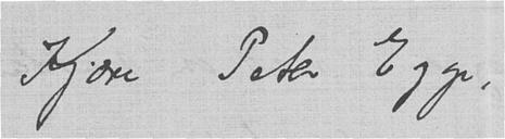Kjære Peter Egge,
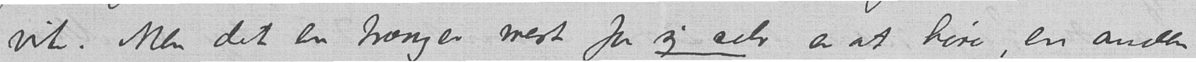vite. Men det en trænger mest for sig selv er at høre, en anden
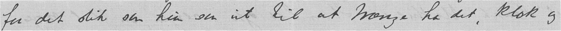faa det slik som hun saa ut til at trænge ha det, klok og
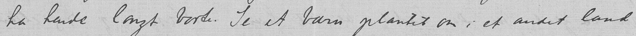ha hende langt borte. Se et barn plantet om i et andet land
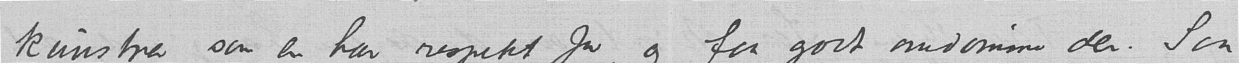kunstner, som en har respekt for, og faa godt omdømme der. Saa
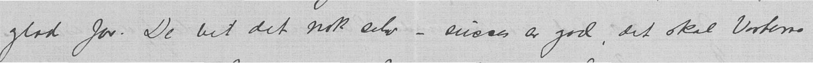glad for. De vet det nok selv – succes er god, det skal Verden
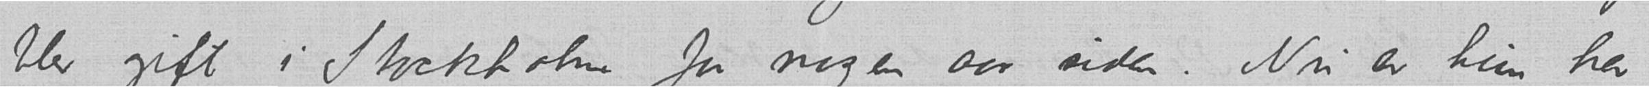blev gift i Stockholm for nogen aar siden. Nu er hun her
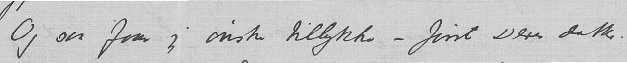Og saa faar jeg ønske tillykke – først Deres datter.
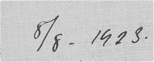8/8-1923.
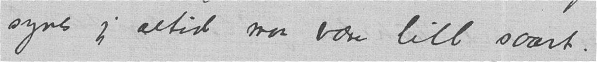synes jeg altid maa være litt saart.
